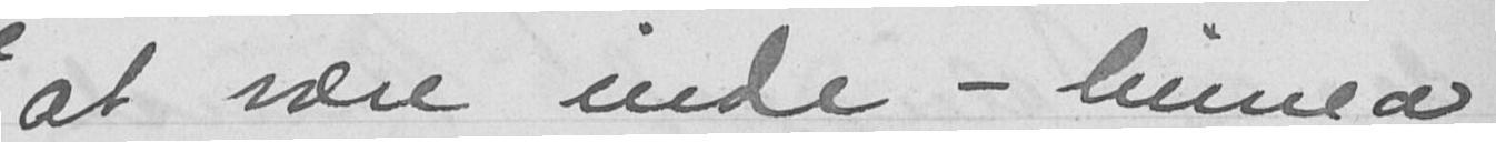at være inde - linnea
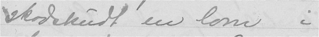skadeskudt en lom i
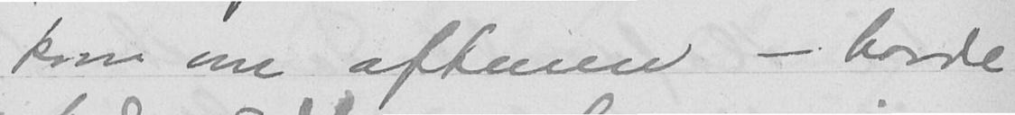kom om aftenen - havde
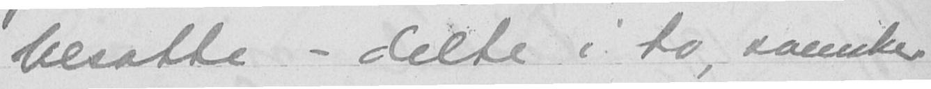besatte - delte i to, svenske
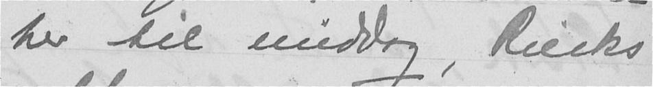her til middag, Riecks
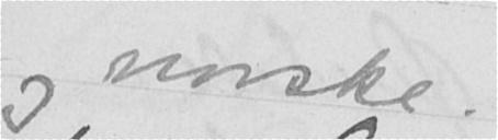og norske.
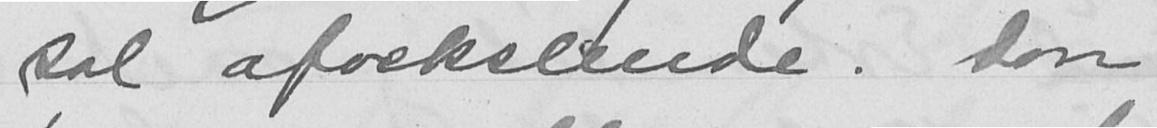sal afvekslende. Don
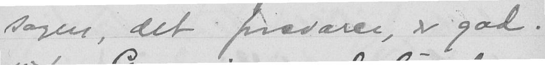sagen, det forsvarer, er god.
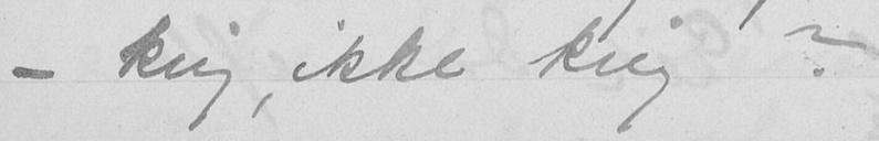- krig, ikke krig?
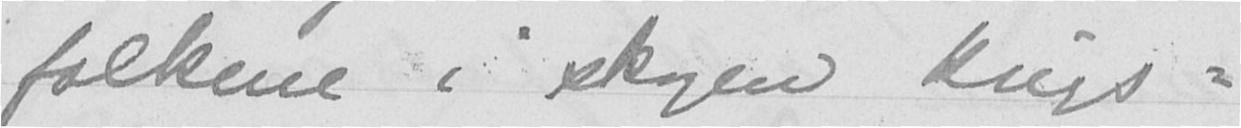folkene i skogen krigs-
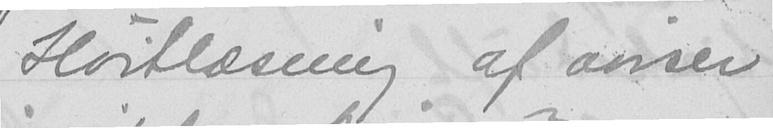Høitlæsning af aviser
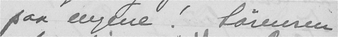paa engene. Sørensen
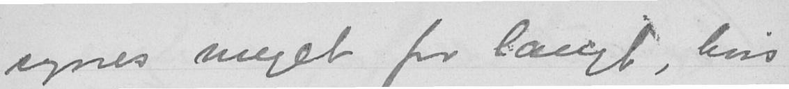synes meget for langt, hvis
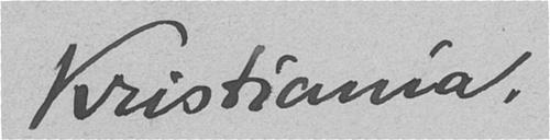Kristiania.
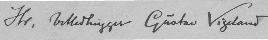Hr. billedhugger Gustav Vigeland
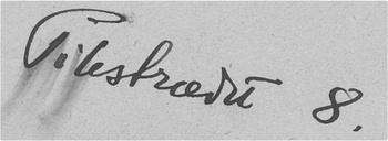Pilestrædet 8.
